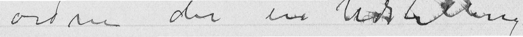ordne der en Udstilling
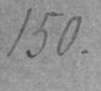150.
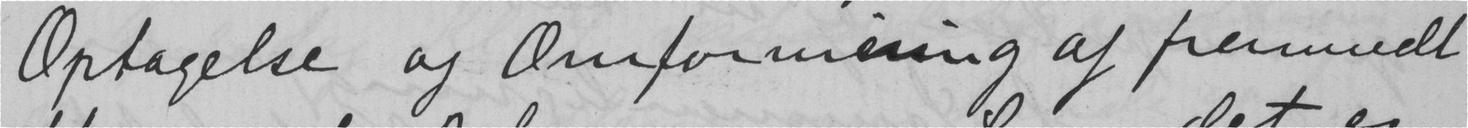Optagelse og Omformning af fremmedt
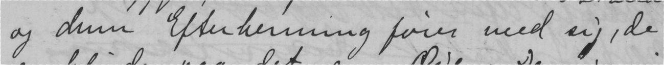og dens Efter henning fører med sig, de
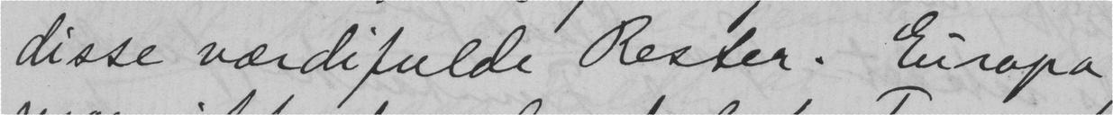disse værdifulde Rester. Europa
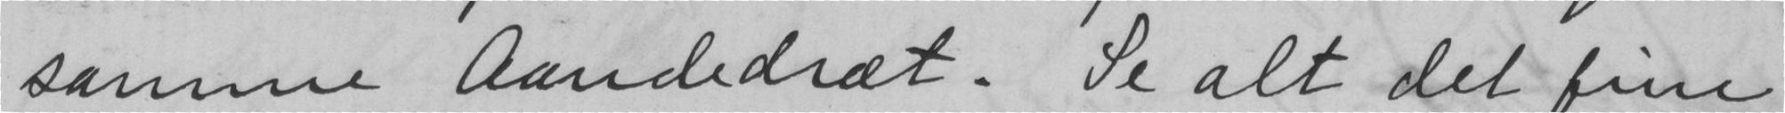samme Aandedræt. Se alt det fine
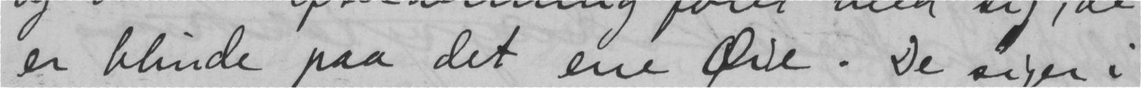er blinde paa det ene Øie. De siger i
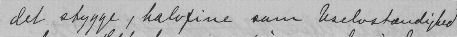det stygge, halvfine som Uselvstændighed
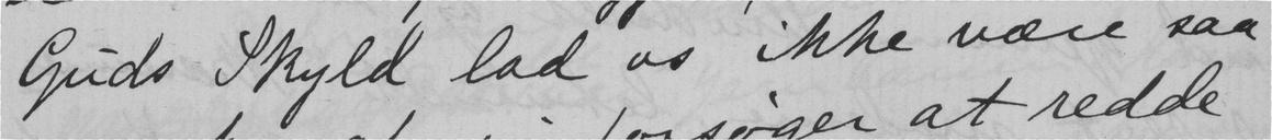Guds Skyld lad os ikke være saa
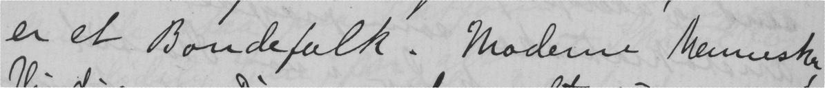er et Bondefolk. Moderne mennesker,
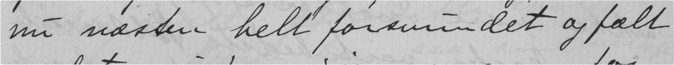nu næsten helt forsvundet og fælt
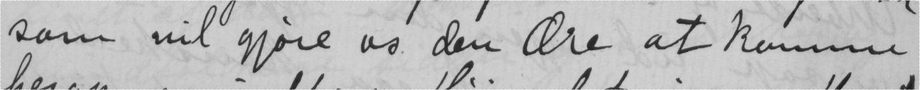som vil gjøre os den ære at komme
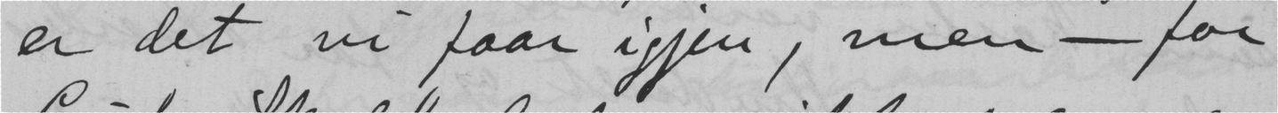er det vi faar igjen, men - for
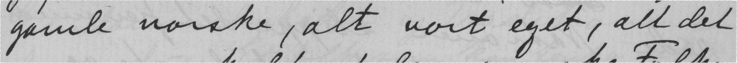gamle norske, alt vort eget, alt det
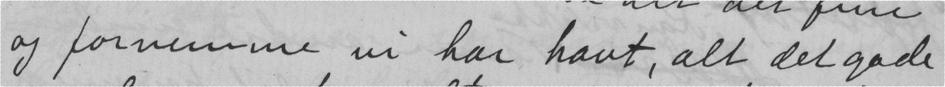og fornemme vi har havt, alt det gode
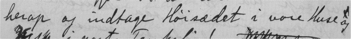herop og indtage Høisædet i vore Huse og
